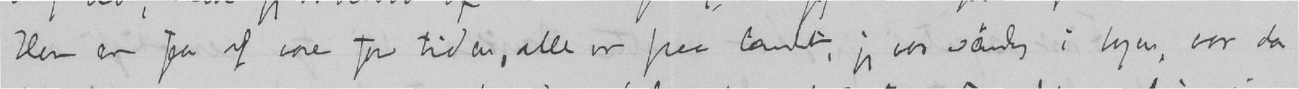Her er faa af vore for tiden, alle er paa landet, jeg bor stadig i byen, bor da
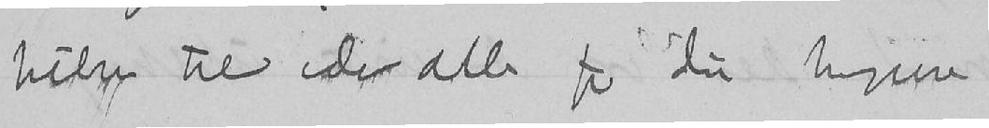hilsen til eder alle fra din hengivne
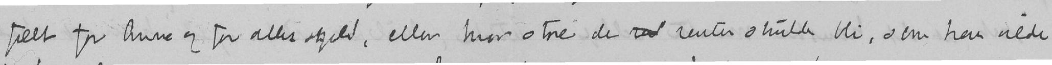fælt for Anna og for alles skyld, eller hvor store de ret renter skulde bli, som han vilde
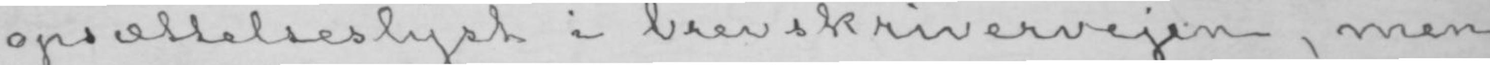opsættelseslyst i brevskrivervejen, men
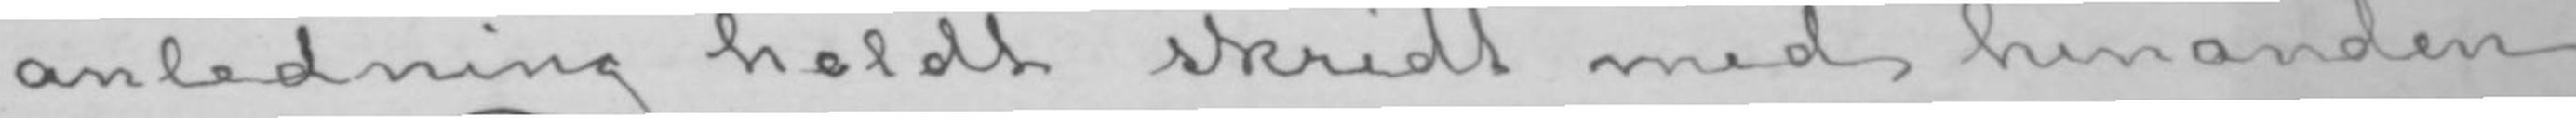anledning holdt skridt med hinanden
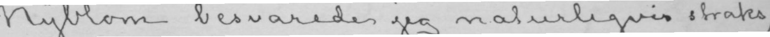Nyblom besvarede jeg naturligvis straks,
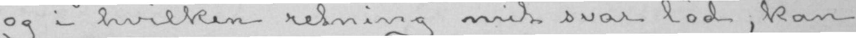og i hvilken retning mit svar lød, kan
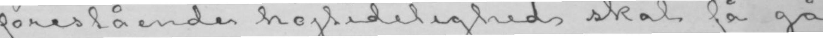forestående højtidelighed skal få gå
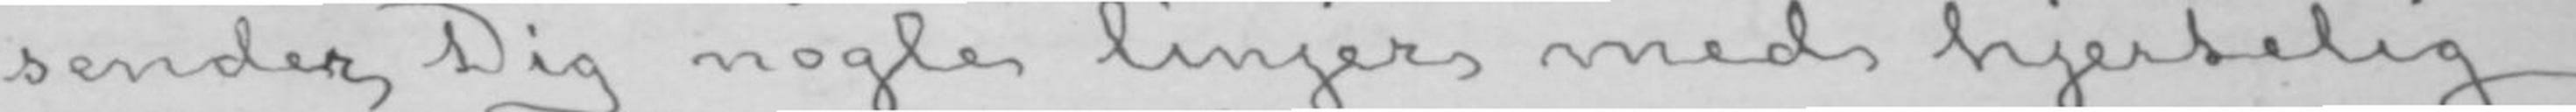sender Dig nogle linjer med hjertelig
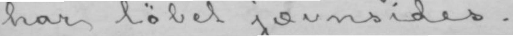har løbet jævnsides.
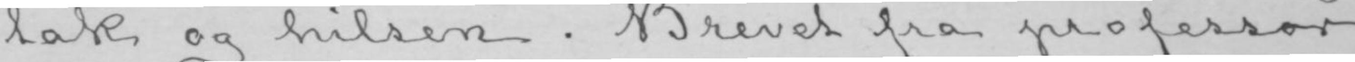tak og hilsen. Brevet fra professor
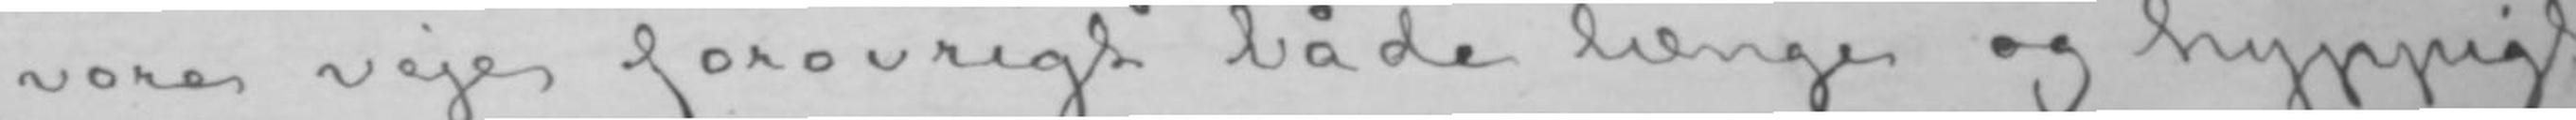vore veje forøvrigt både længe og hyppigt
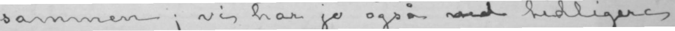sammen; vi har jo også ved tidligere
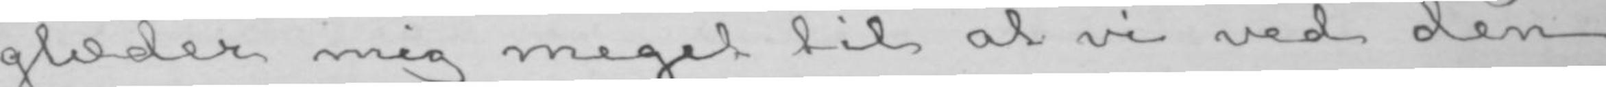glæder mig meget til at vi ved den
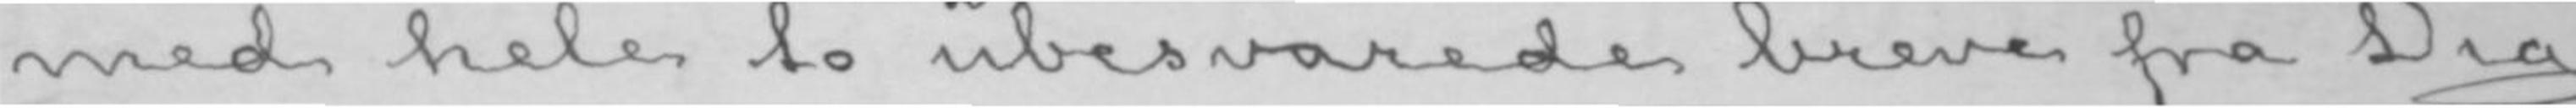med hele to ubesvarede breve fra Dig,
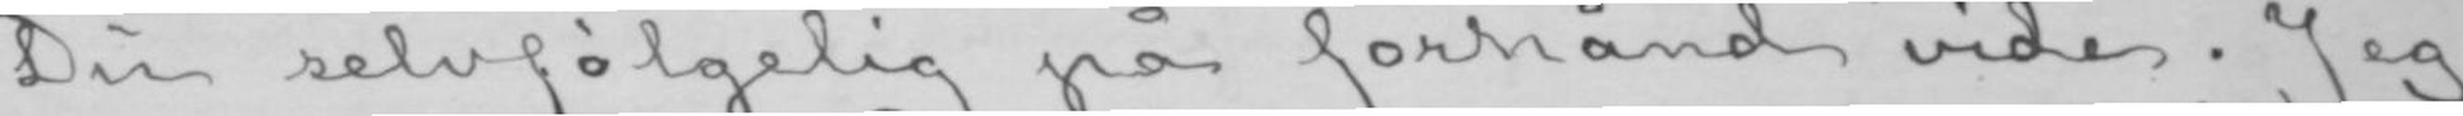Du selvfølgelig på forhånd vide. Jeg
